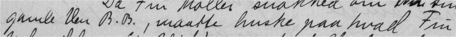gamle ven B. B., maatte huske paa hvad Fru
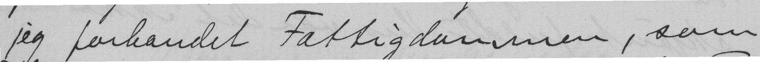jeg forbandet Fattigdommen, som
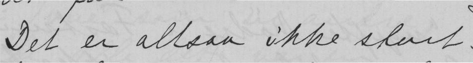Det er altsaa ikke stort
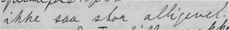ikke saa stor alligevel.
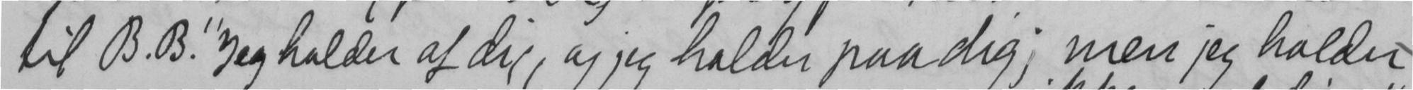til B B. "Jeg holder af dig, og jeg holder paa dig; men jeg holder
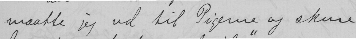maatte jeg ud til Pigerne og skure
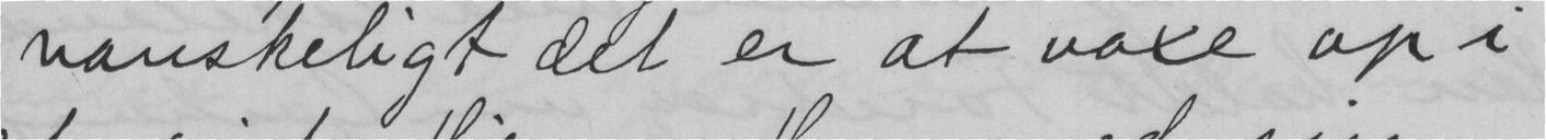vanskeligt det er at voxe op i
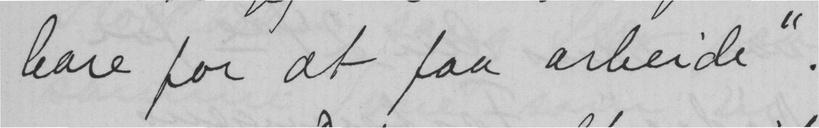bare for at faa arbeide".
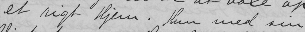et rigt Hjem. Hun med sin
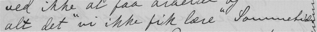alt det "vi ikke fik lære" Sommetider
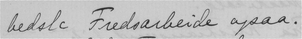bedste Fredsarbeide ogsaa.
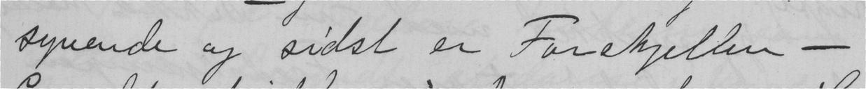syvende og sidst er Forskjellen -
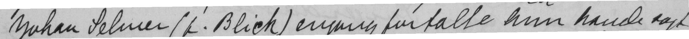Johan Selmer (f. Blick) engang fortalte hun havde sagt
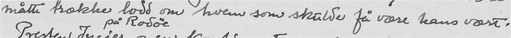måtte trække lodd om hvem som skulde få være hans vært.
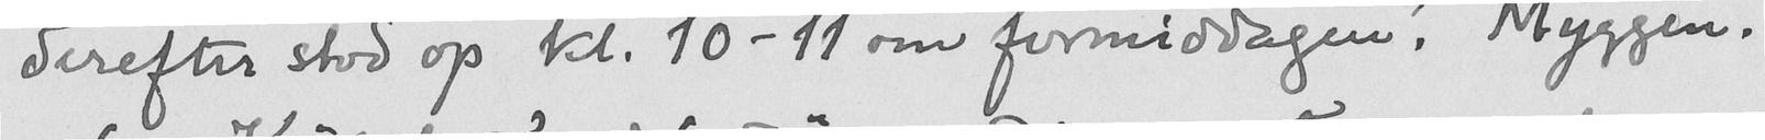derefter stod op kl. 10 - 11 om formiddagen." Myggen.
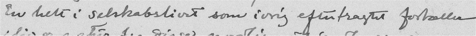En helt i selskabslivet som ivrig eftertragtet fortæller
